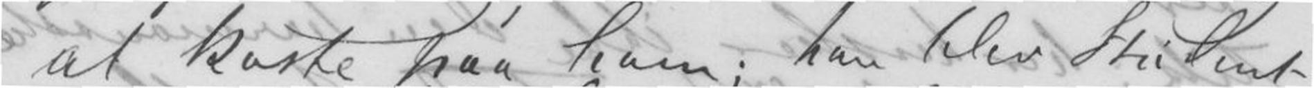at koste paa ham; han blev Student
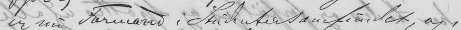er nu Formand i Studentersamfundet, og i
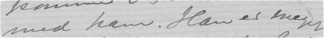med ham. Han er meget
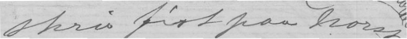skriv først paa Norsk
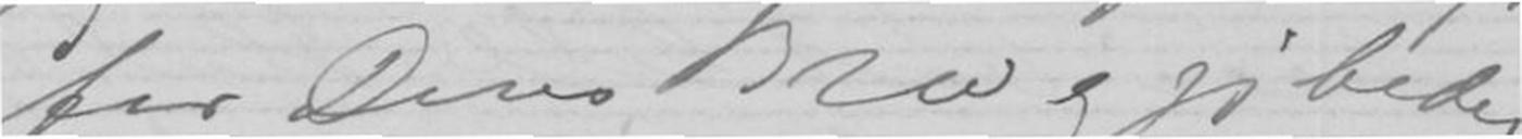for Deres Brev og jeg beder
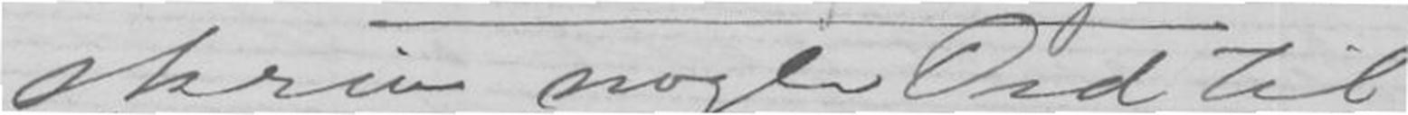skrive nogle Ord til
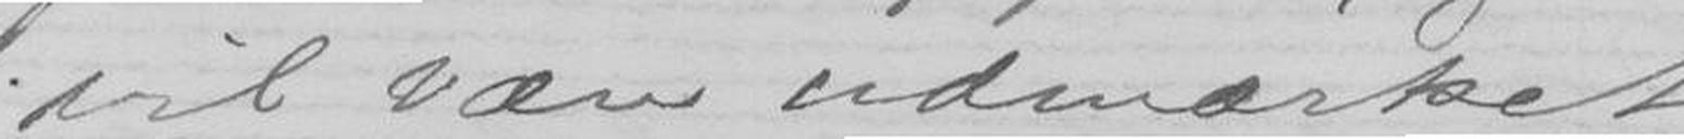vil være udmærket.
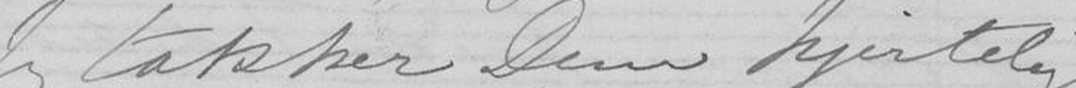Jeg takker Dem hjerteligt
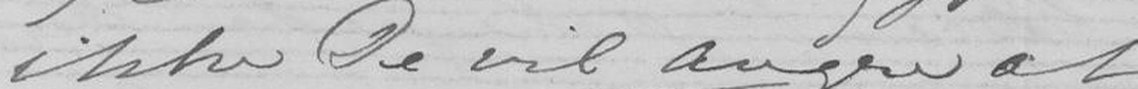ikke De vil angre at
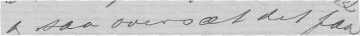og saa oversæt det paa
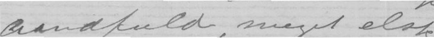aandfuld, meget elsk
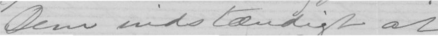Dem indstændigt at
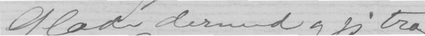Glæde dermed og jeg tror
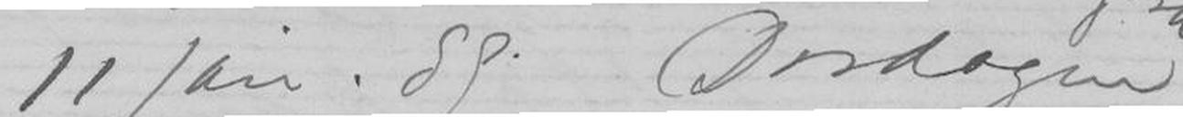11 jan. 89Dordogne
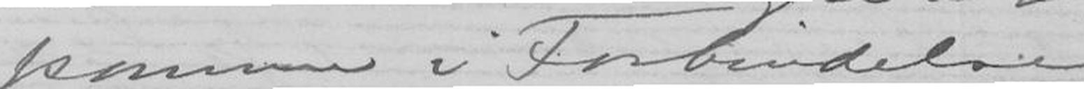komme i Forbindelse
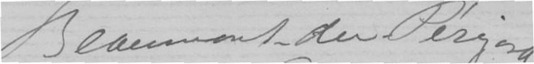Beaumont-du-Périgord
